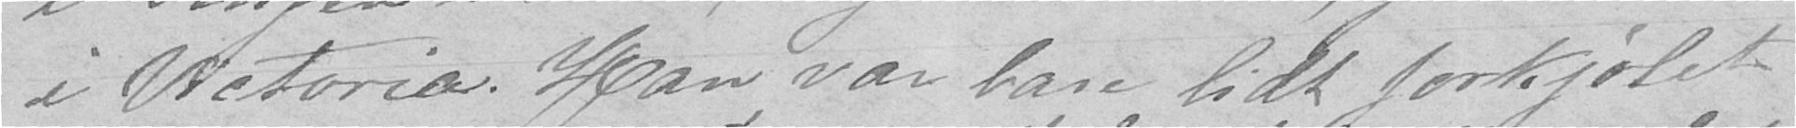i Victoria. Han var bare lidt forkjølet
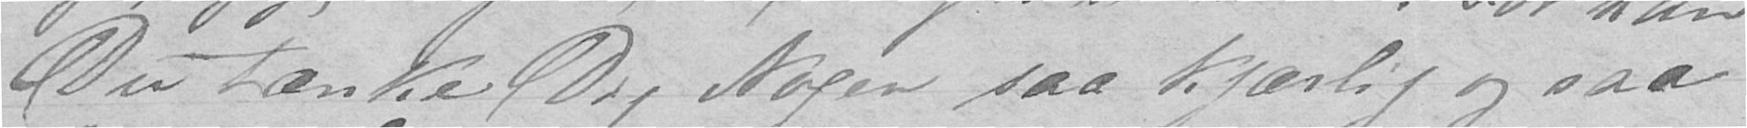Du tænke Dig Nogen saa Kjærlig og saa,
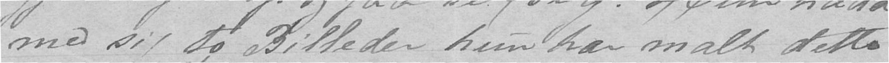med sig to Billeder hun har malt dette
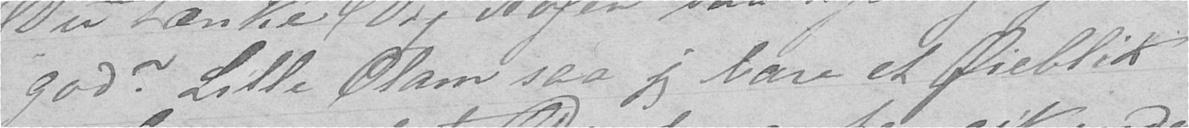god? Lille Olam saa jeg bare et Øieblik
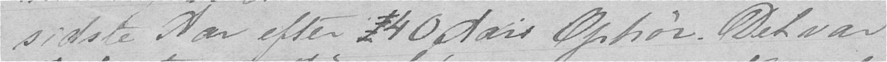sidste Aar efter 140 Aars Ophor. Det var
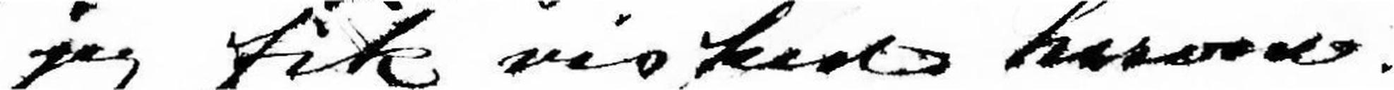jeg fik vished herved!
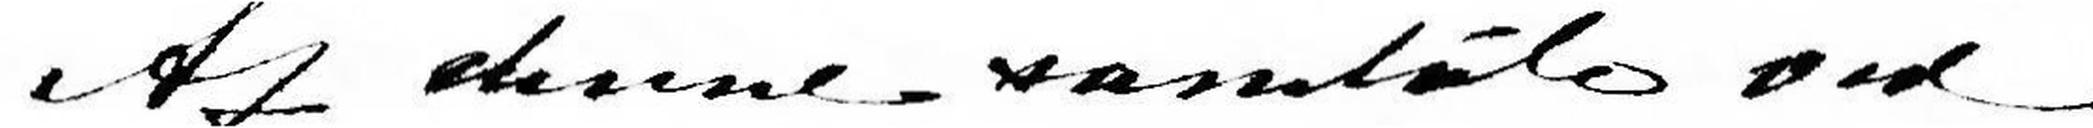Af denne samtale ved
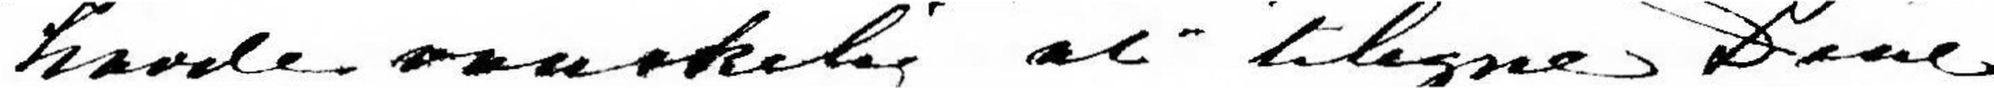havde vanskelig at tilegne Dem
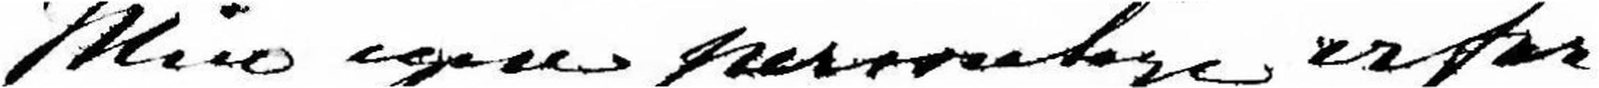Min egen personlige erfar-
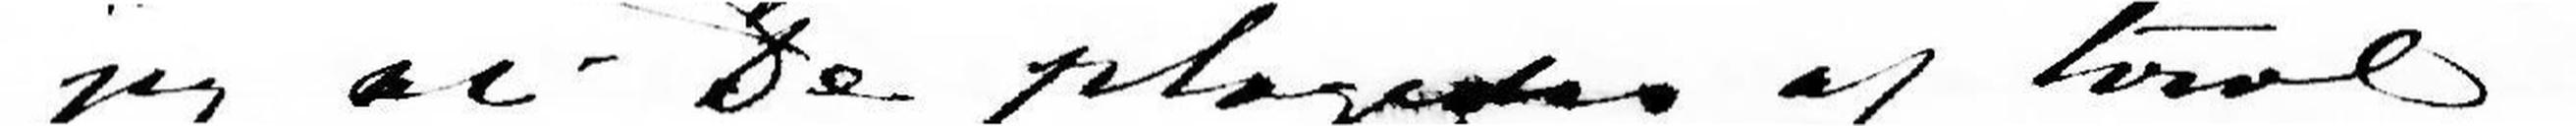jeg at De plagedes af tvivl og
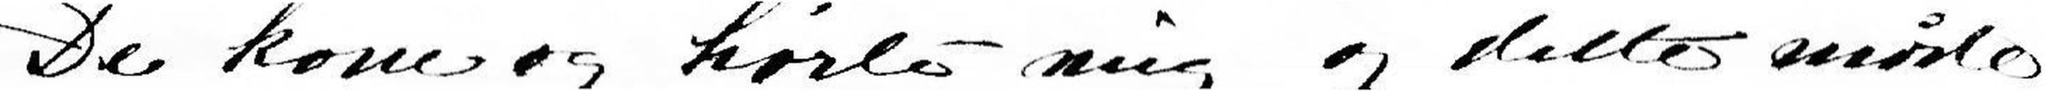men De kom og hørte mig og dette møde
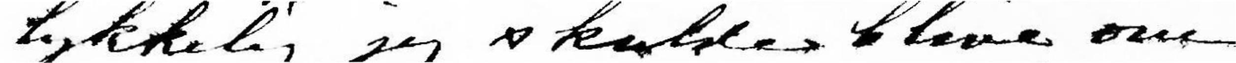lukkelig jeg skulde blive om
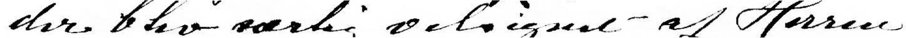der blev velsignet af Herren
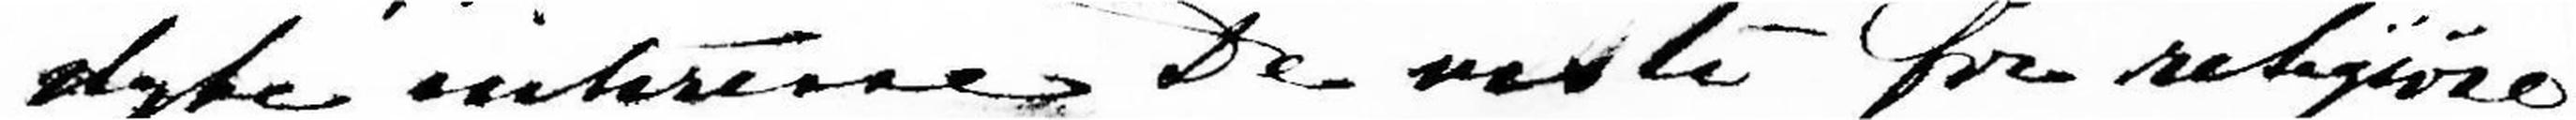dybe intresse De viste for religiøse
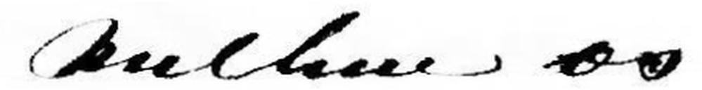mellem os.
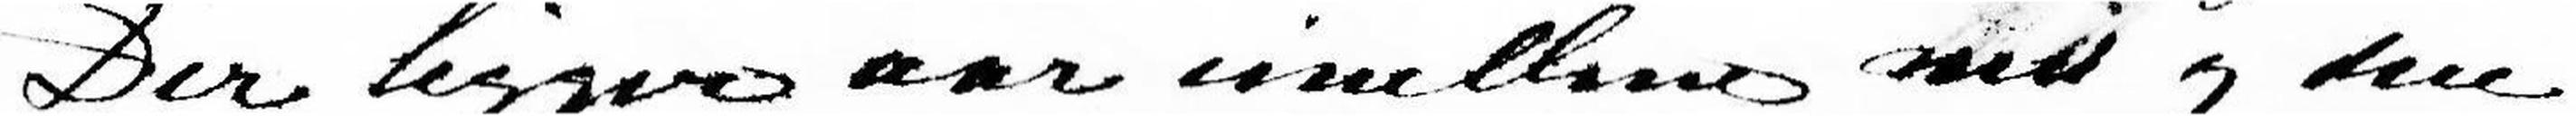Der ligger aar imellem mit og den
